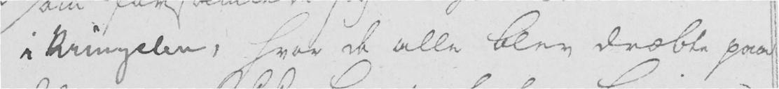i Kringelen, hvor de alle blev dræbte paa
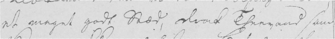et meget godt Stæd, drak Theevand som
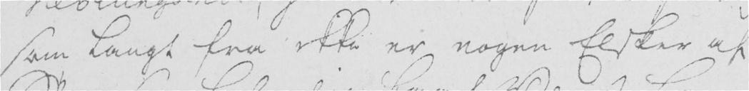som langt fra ikke er nogen elsker af
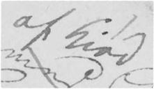af kiød
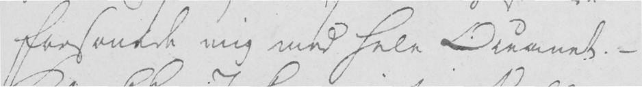forsonede mig med hele Oceanet. -
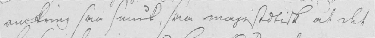omkring saa smuck, saa majestætisk at det
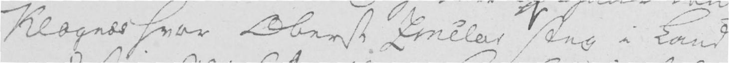Klognæs hvor Oberst Zinclar steg i Land
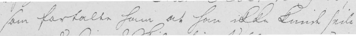som fortalte ham at han ikke kunde seile
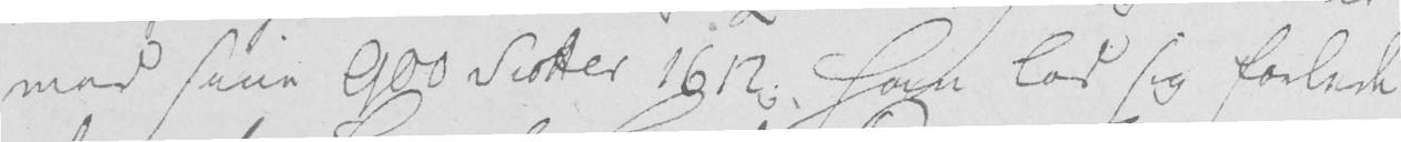med sine 900 Scotter 1612; han lod sig forlede
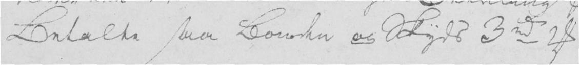Betalte saa Bonden os Skyds 3 rd 2 s
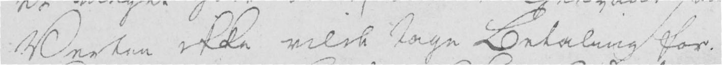Verten ikke vilde tage Betaling for.
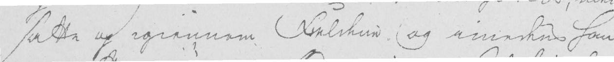satte op igiennem Fieldene og imedens han
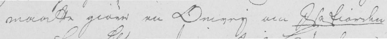maatte giøre en Omvej om Isefiorden
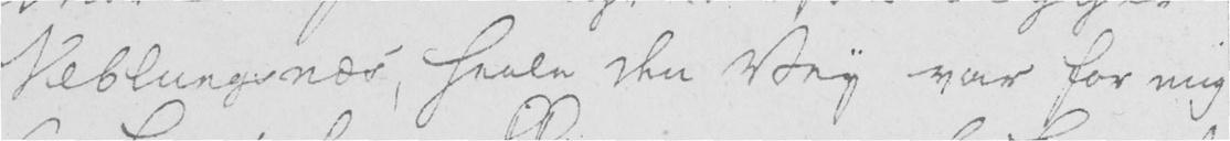Veblungsnæs, heele den Vey var for mig
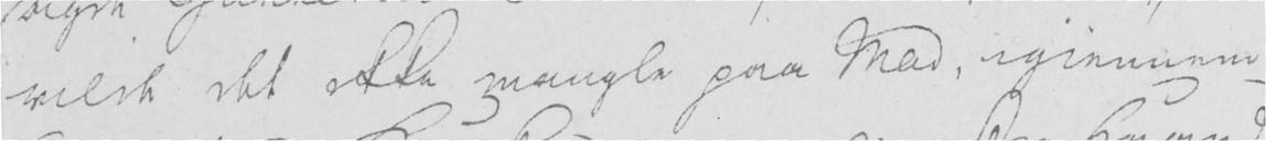vilde det ikke mangle paa Mad, igiennem
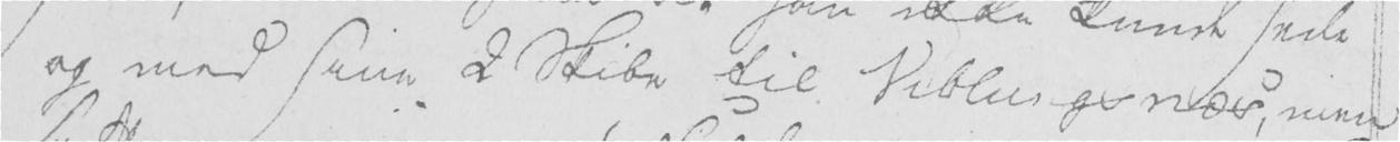op med sine 2 Skibe til Veblungsnæs, men
